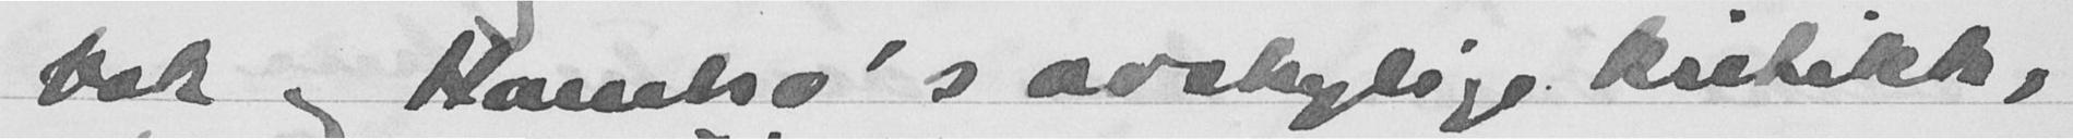bok og Hambro's avskylige kritikk,
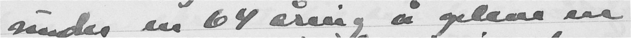under en 64 åring å opleve en
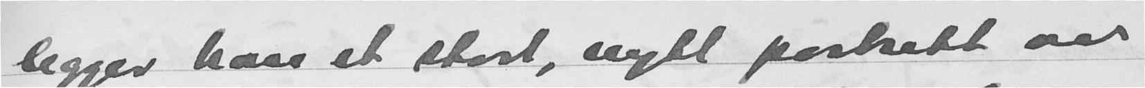legger han et stort, nytt portrett av
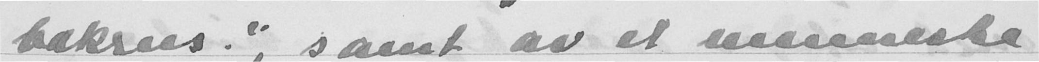bakrus.", samt av et menneske
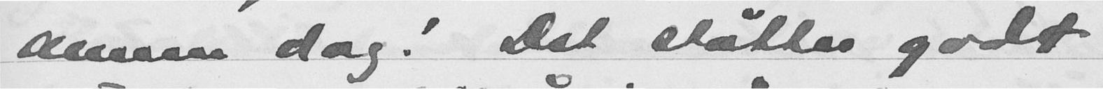annen dag. Det støtter godt
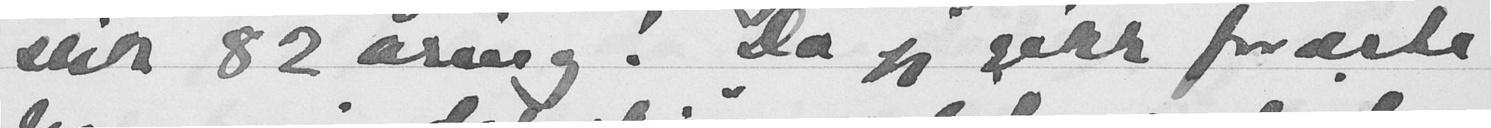slik 82 åring. Da jeg gikk forærte
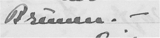Brunen. -
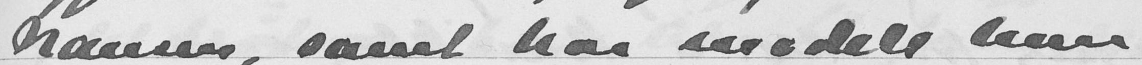Nansen, samt har modell hver
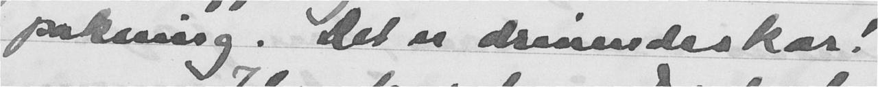pakning. Det er drivendes kar!
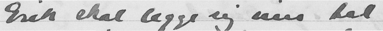Erik skal legge sig inn til
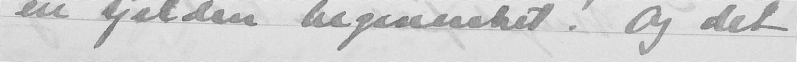en sjelden begivenhet! Og det
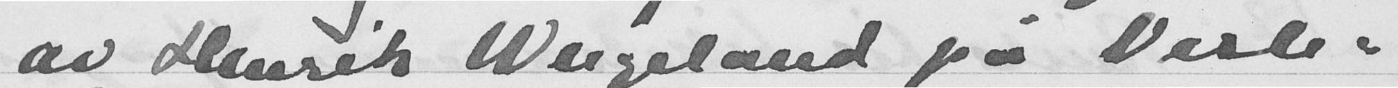av Henrik Wergeland på Vesle-
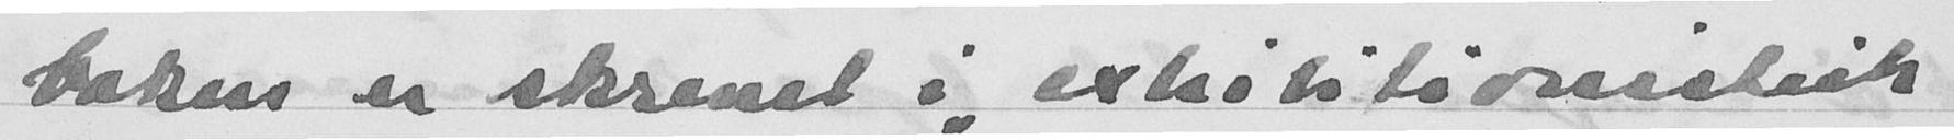boken er skrevet i "exhibitionistisk
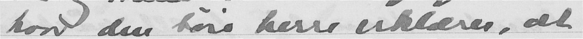hvor den høie herre erklærer, at
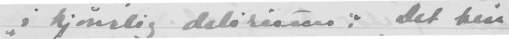"i kjønslig delirium:" Det blir
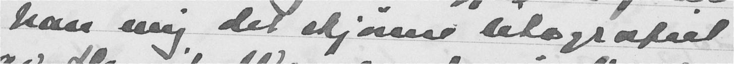han mig det skjønne litografiet
